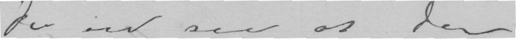du vil see at Du
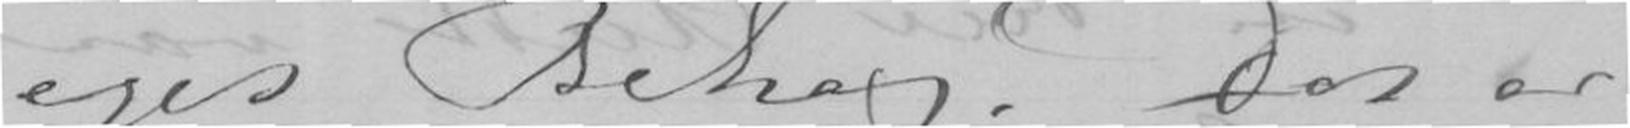eget Behag. Det er
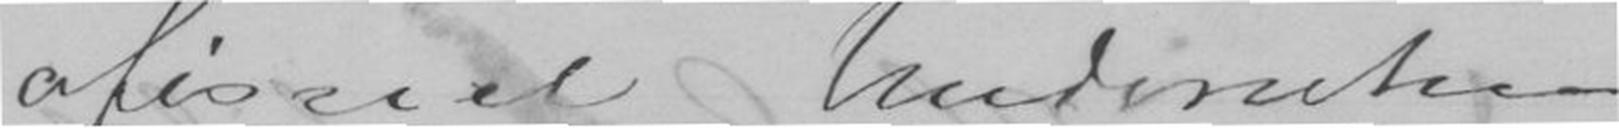oficiel Underretning
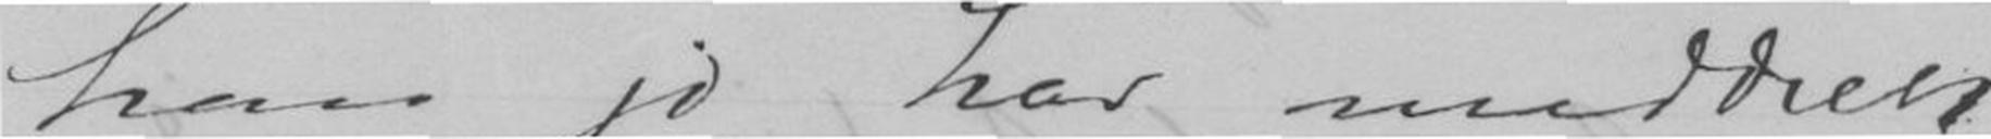han jo har meddeelt
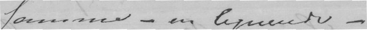Samme - en lignende -
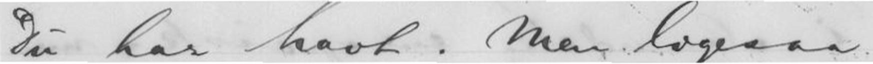Du har havt. Men ligesaa
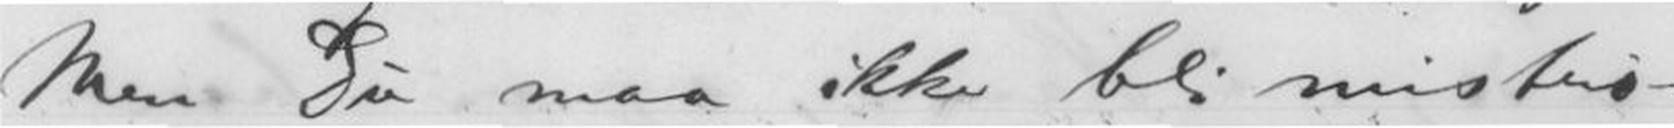Men Du maa ikke bli mistrø-
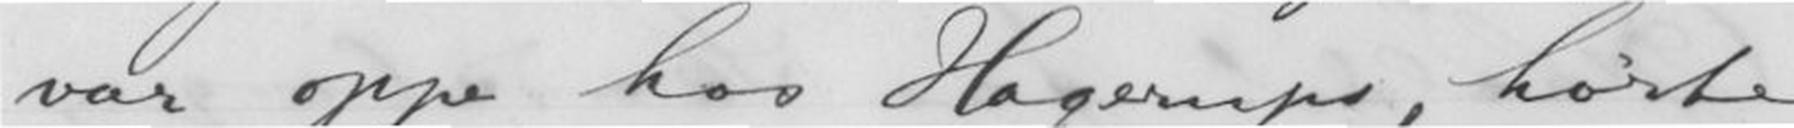var oppe hos Hagerups, hørte
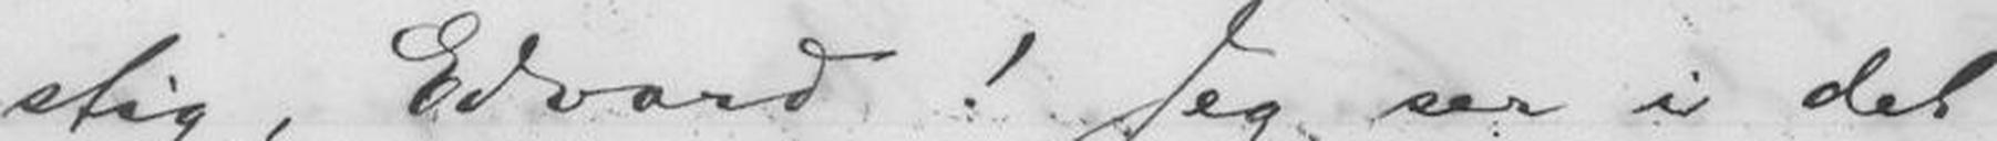stig, Edvard! Jeg ser i det
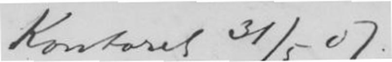Kontoret 31/5 07
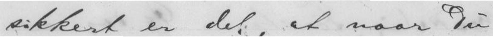sikkert er det, at naar Du
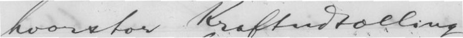hvorstor Kraftudtælling
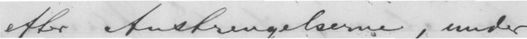efter Anstrengelserne, under
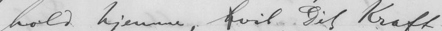hold hjemme, hvil Dit Kraft-
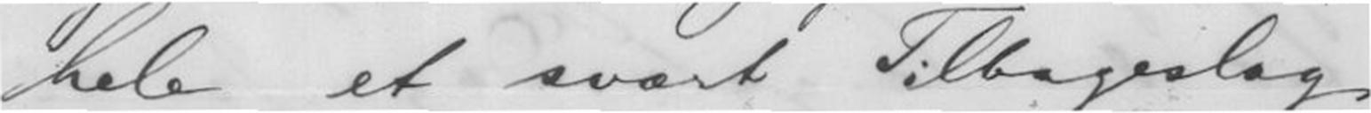hele et svært Tilbageslag
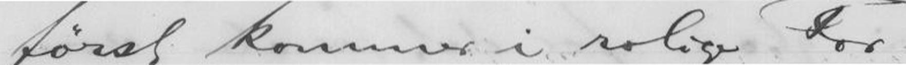først kommer i rolige For-
